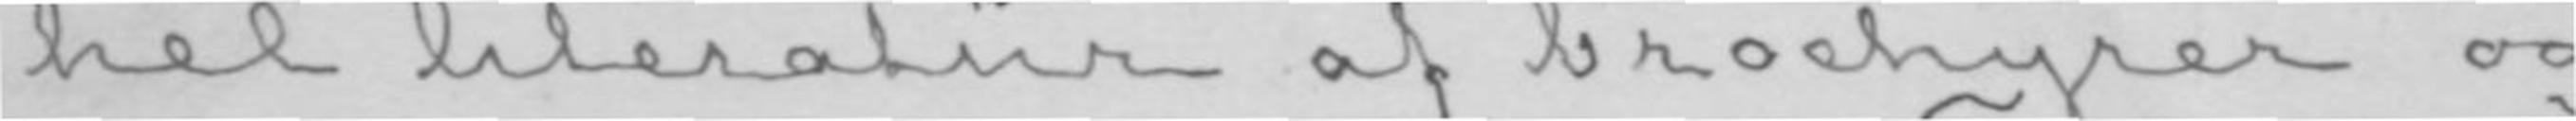hel literatur af brochyrer og
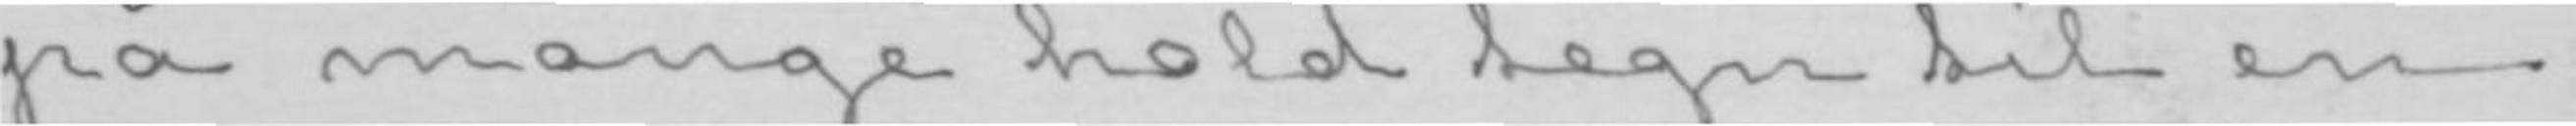på mange hold tegn til en
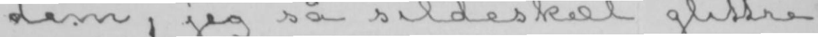dem;, jeg så sildeskæl glittre
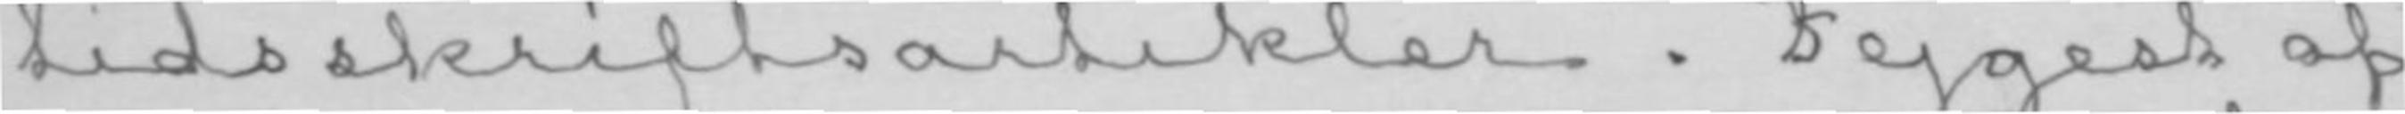tidsskriftartikler. Fejgest af
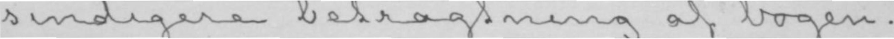sindigere betragtning af bogen.
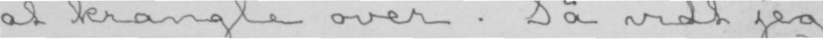at krangle over. Så vidt jeg
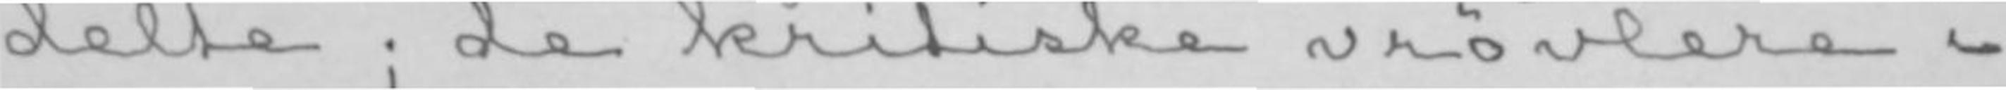delte; de kritiske vrøvlere i
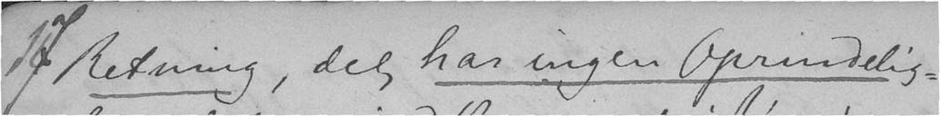Retning, det har ingen Oprindelig-
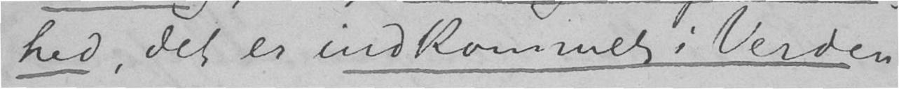hed, det er indkommelt i Verden
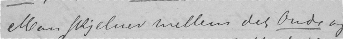Man skjelner mellem det Onde og
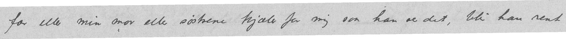far eller min mor eller søstrene kjæler for mig som han ser det, blir han rent
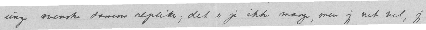unge svenske damens repliker, det er jo ikke mange, men jeg vet vel, jeg
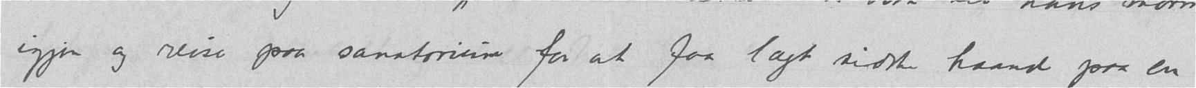igjen og reise paa sanatorium for at faa lagt sidste haand paa en
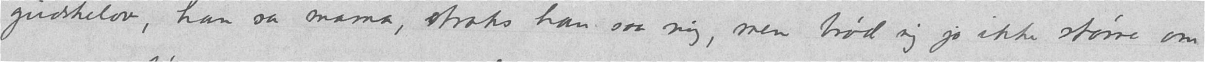gudskelov, han sa mama, straks han saa mig, men brød sig jo ikke større om
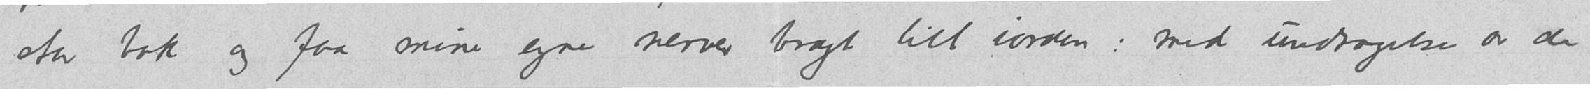stor bok og faa mine egne nerver bragt litt iorden: med undtagelse av de
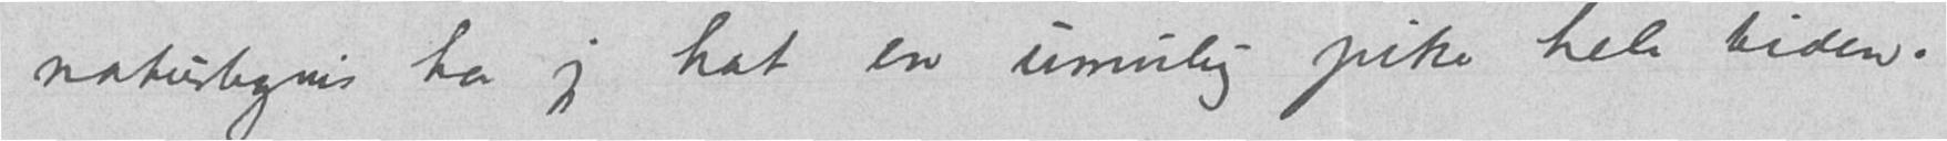naturligvis har jeg hat en umulig pike hele tiden.
